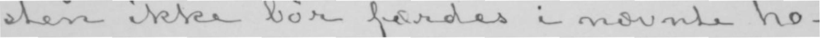sten ikke bør færdes i nævnte ho-
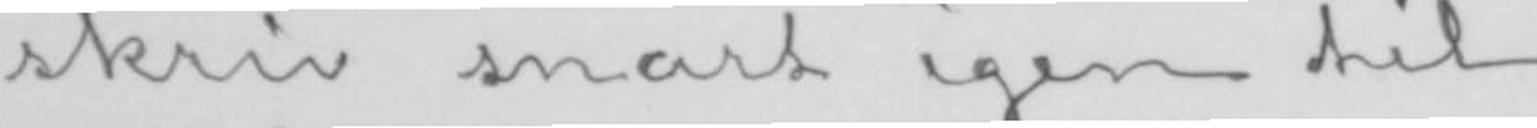skriv snart igen til
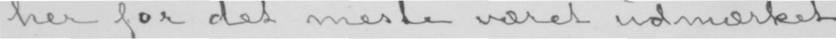her for det meste været udmærket
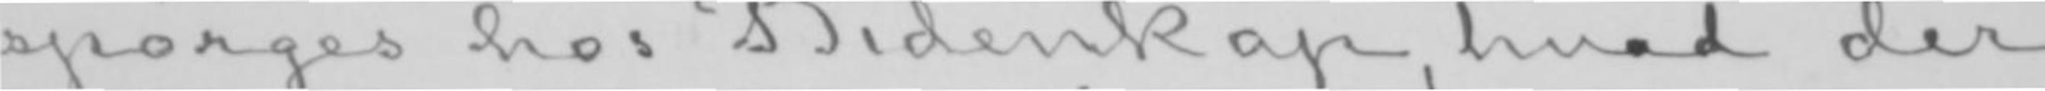spørges hos Bidenkap, hvad der
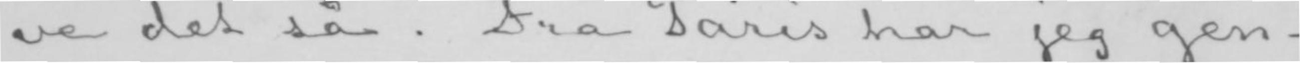ve det så. Fra Paris har jeg gen-
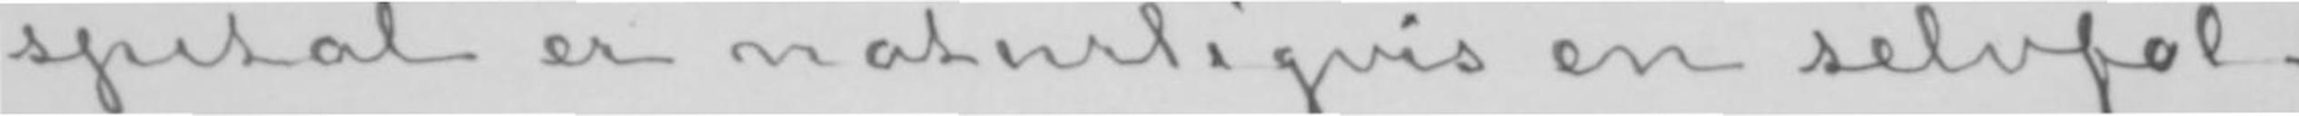spital er naturligvis en selvføl-
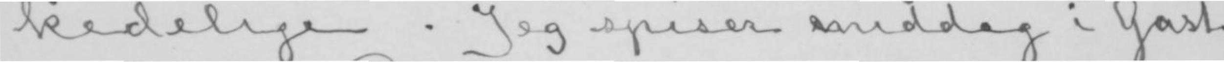kedelige. Jeg spiser imiddag i Gast-
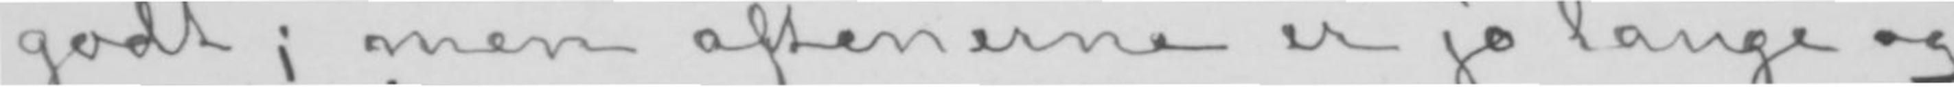godt; men aftenerne er jo lange og
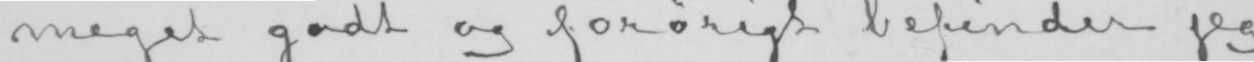meget godt og forørigt befinder jeg
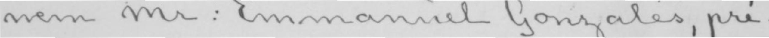nem Mr: Emmanuel Gonzalés, pré-
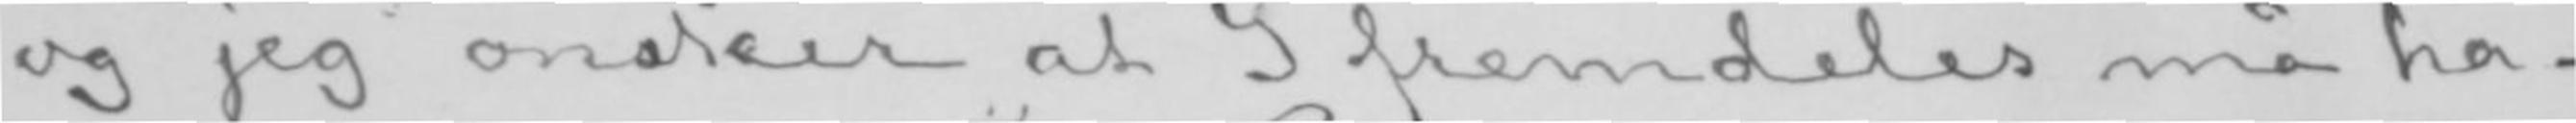og jeg ønsker at I fremdeles må ha-
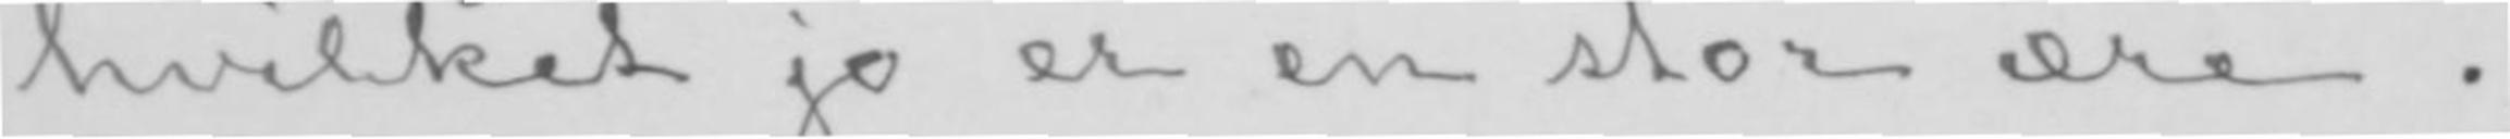hvilket jo er en stor ære.
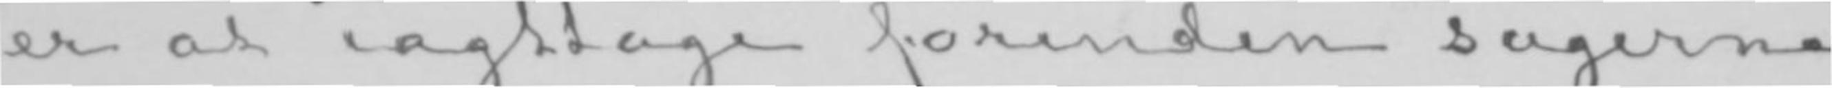er at iagttage forinden sagerne
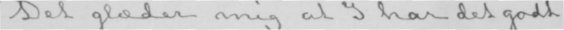Det glæder mig at I har det godt,
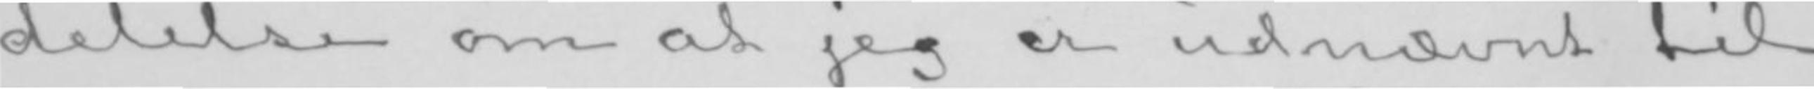delelse om at jeg er udnævnt til
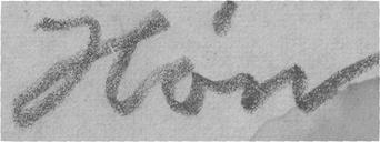Høn
